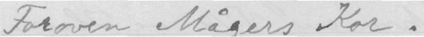Foroven Mågers Kor.
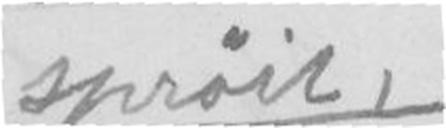sprøit,
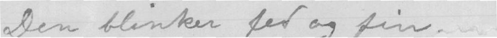Den blinker fed og fin.
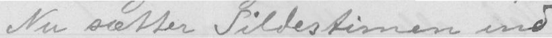Nu sætter Sildestimen ind
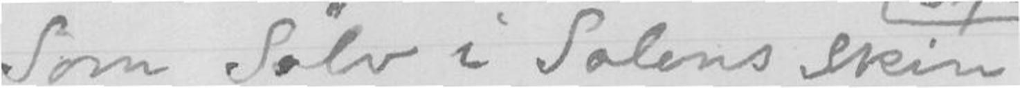Som Sølv i Solens Skin
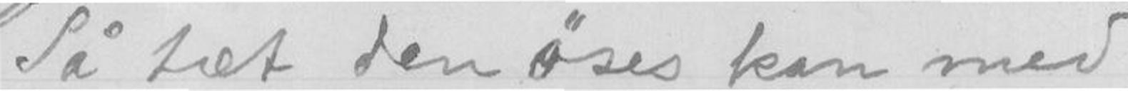Så tæt den øses kan med
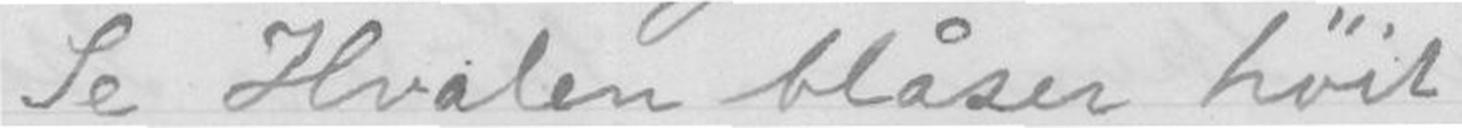Se Hvalen blåser høit
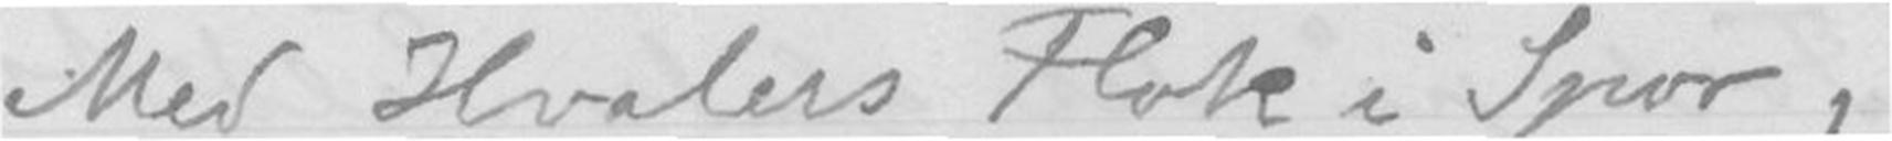Med Hvalers Flok i Spor,
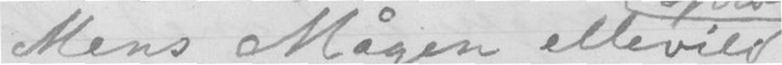Mens Mågen ellevild
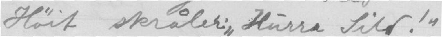Høit skråler: "Hurra Sild!"
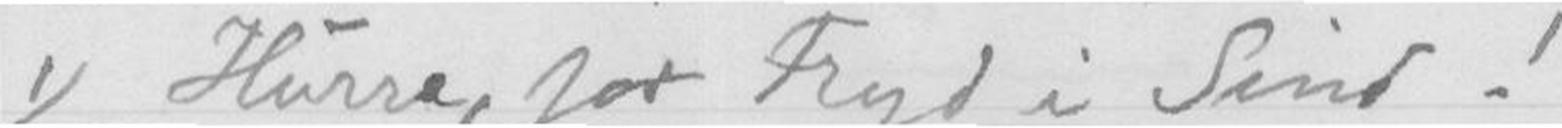1) Hurra, for Fryd i Sind!
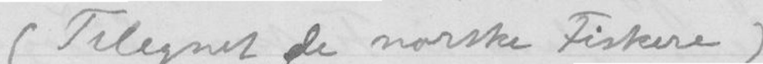(Tilegnet de norske Fiskere)
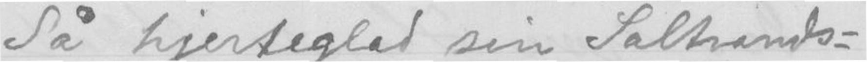Så hjerteglad sin Saltvands-
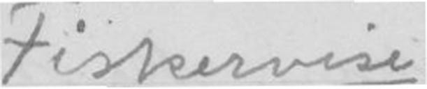* Fiskervise
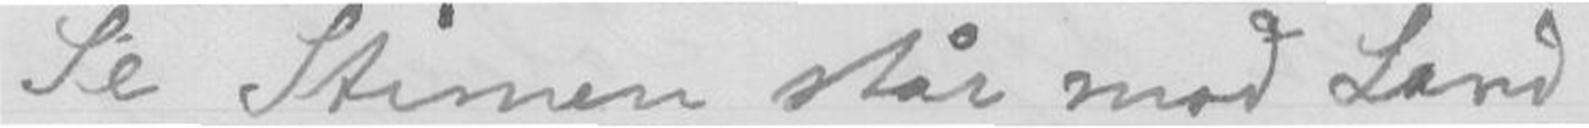Se Stimen står mod Land.
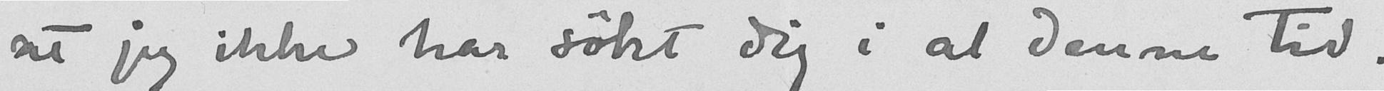at jeg ikke har søkt dig i al denne tid.
